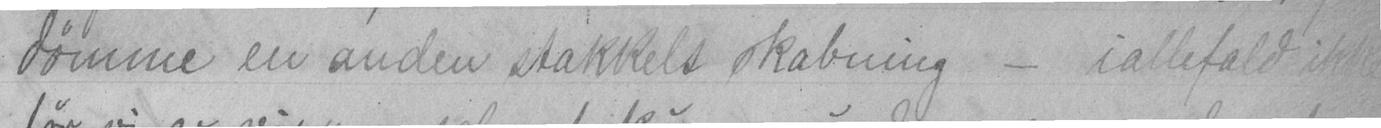dømme en anden stakkels skabning - iallefald ikke
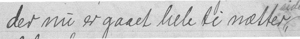der nu er gaaet hele ti nætter
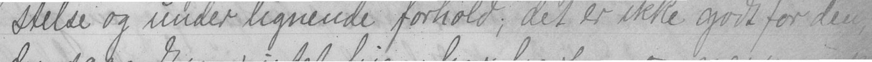stelse og under lignende forhold; det er ikke godt for den
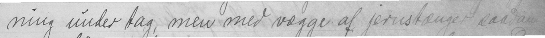ning under tag, men med vægge af jernstænger saadan
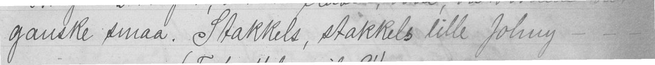ganske smaa. Stakkels, stakkels lille Johny - - -
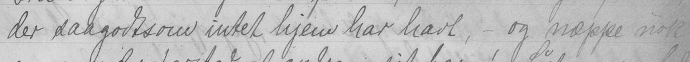der saagodtsom intet hjem har havt, - og næppe nok
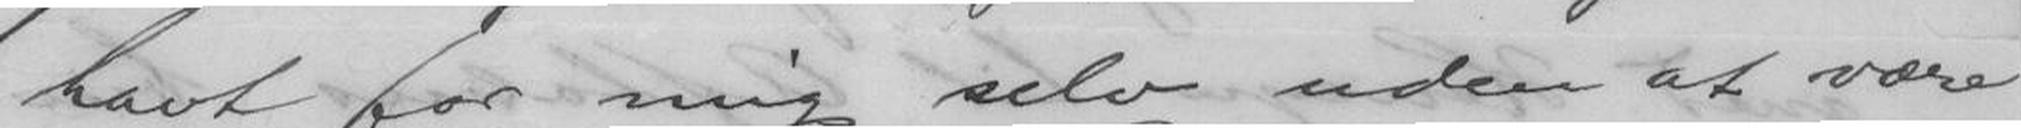havt for mig selv uden at være
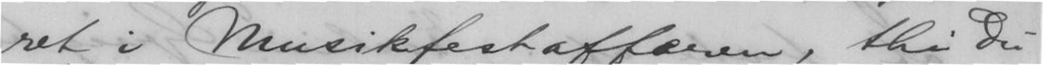ret i Musikfestaffæren, thi Du
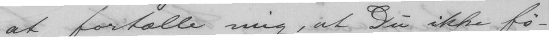at fortælle mig, at Du ikke fø-
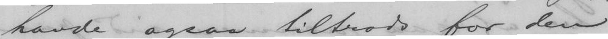havde ogsaa tiltrods for den
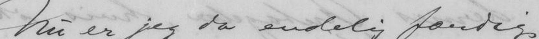Nu er jeg da endelig færdig
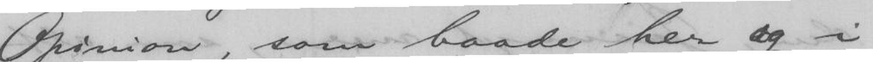Opinion, som baade her og i
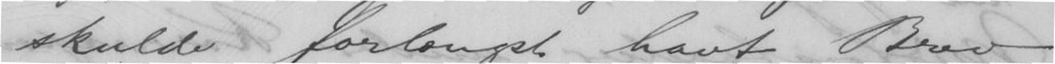skulde forlængst havt Brev
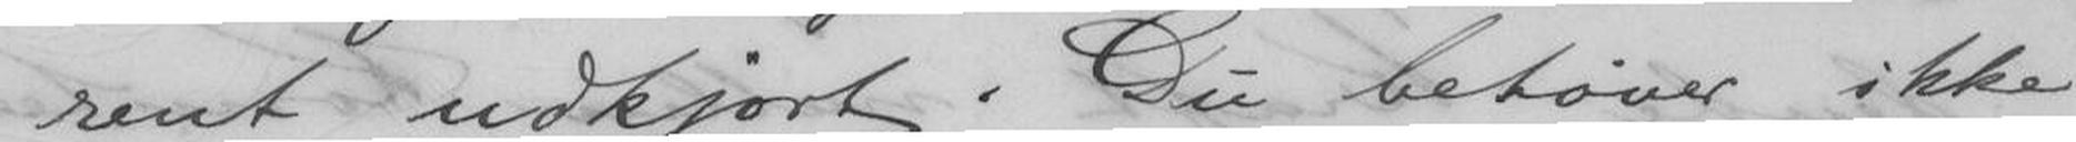rent udkjørt. Du behøver ikke
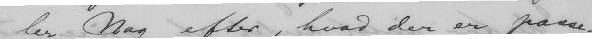ler Nag efter, hvad der er passe-
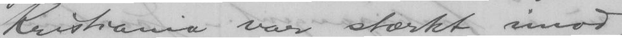Kristiania var stærkt imod,
